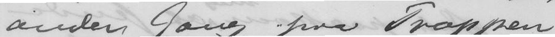anden Gang fra Trappen
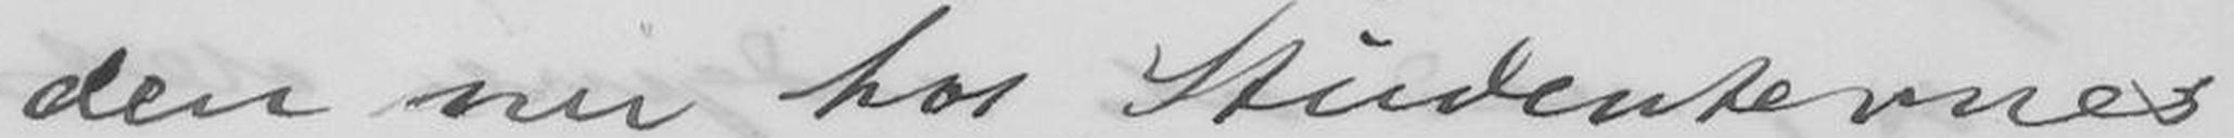den nu hos Studenternes
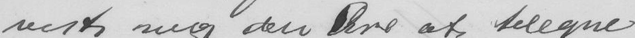vist mig den Ære at tilegne
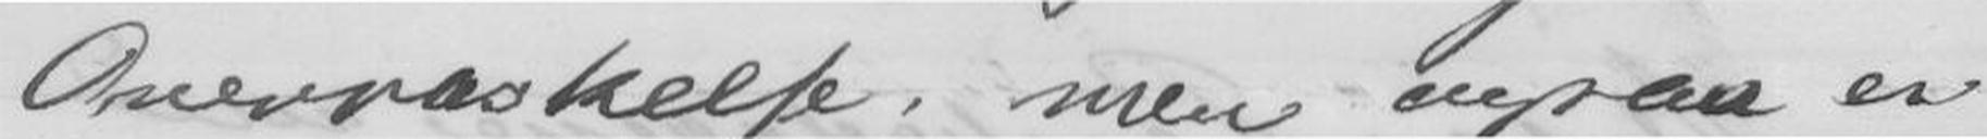Overraskelse, men ogsaa er
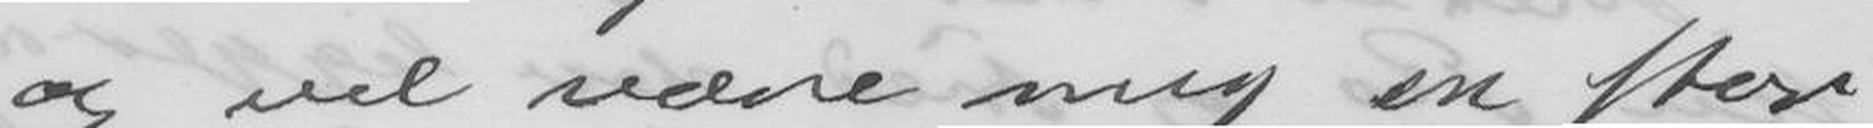og vil være mig en stor
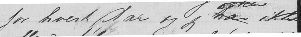for hvert Aar og jeg har ikke
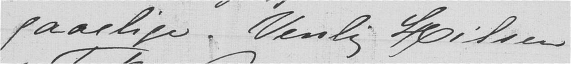gaaelige. Venlig Hilsen
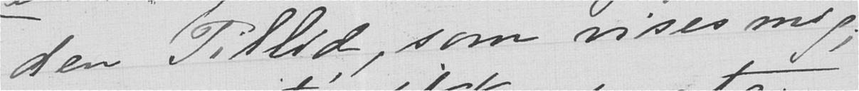den Tillid, som vises mig,
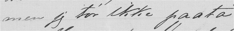men jeg tør ikke paata
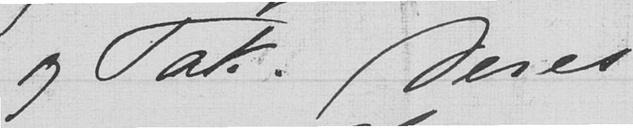og Tak. Deres
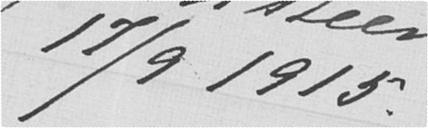17/9 1915.
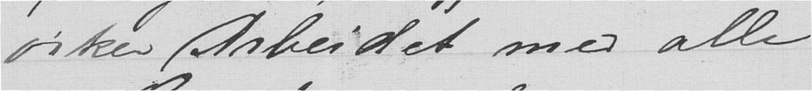viker Arbeidet med alle
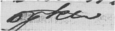orker
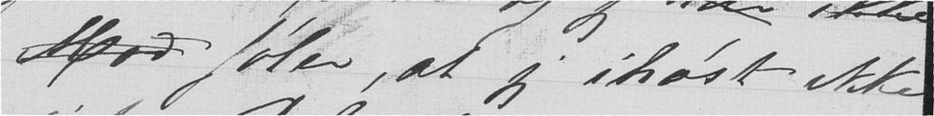Mod føler, at jeg ihøst ikke
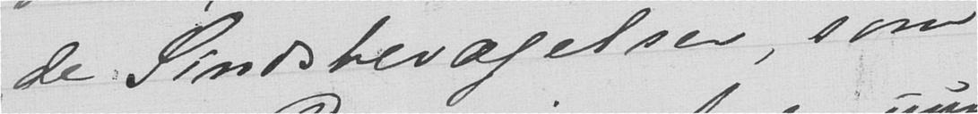de Sindsbevægelser, som
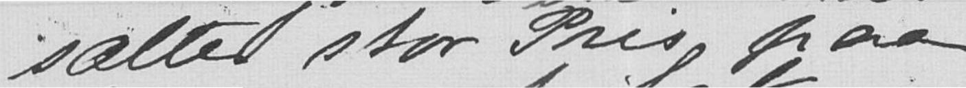sætter stor Pris paa
